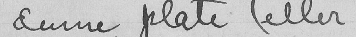denne plate (eller
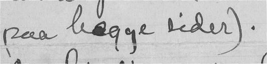paa bægge sider).
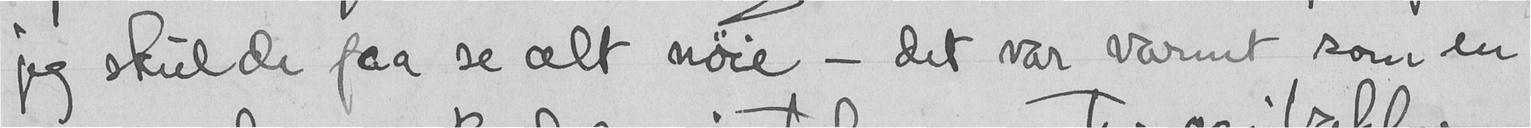jeg skulde faa se alt nøie - det var varmt som en
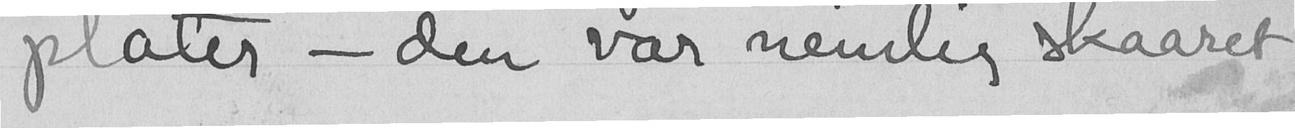plater - den var nemlig skaaret
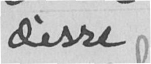disse
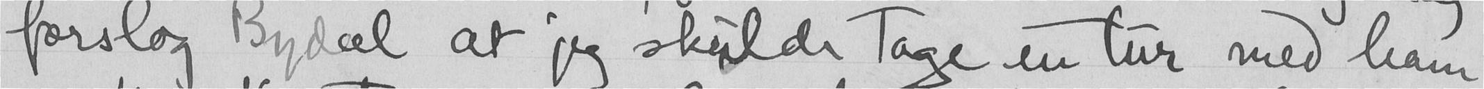forslog Bydal at jeg skulde tage en tur med ham
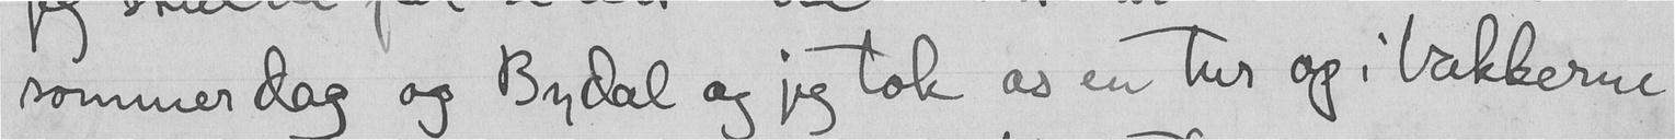sommerdag og Bydal og jeg tok os en tur op i bakkerne
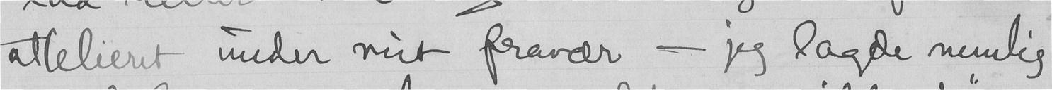attelieret under mit fravær - - jeg lagde nemlig
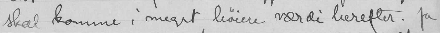skal komme i meget heveere værde herefter. Ja
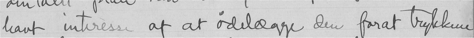havt interesse af at ødelægge den for at trykkene
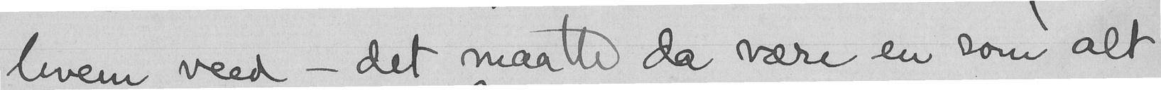hvem veed - det maatte da være en som alt
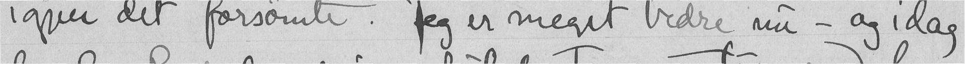igjen det forsømte. Jeg er meget bedre nu - og idag
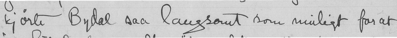kjørte Bydal saa langsomt som muligt for at
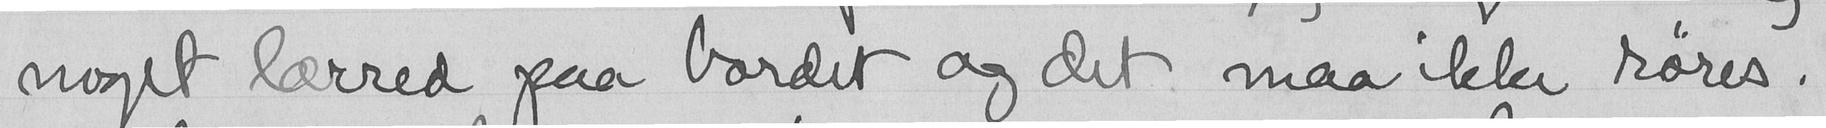noget lærred paa bordet og det maa ikke røres.
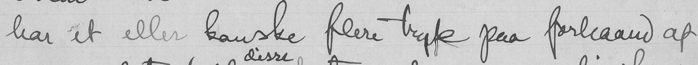har et eller kanske flere tryk paa forhaand af
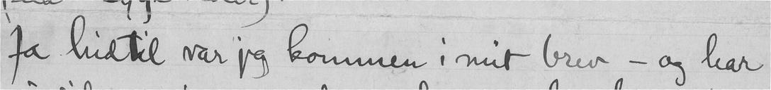Ja hidtil var jeg kommen i mit brev - og har
